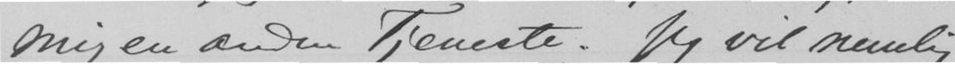mig en anden Tjeneste. Jeg vil nemlig
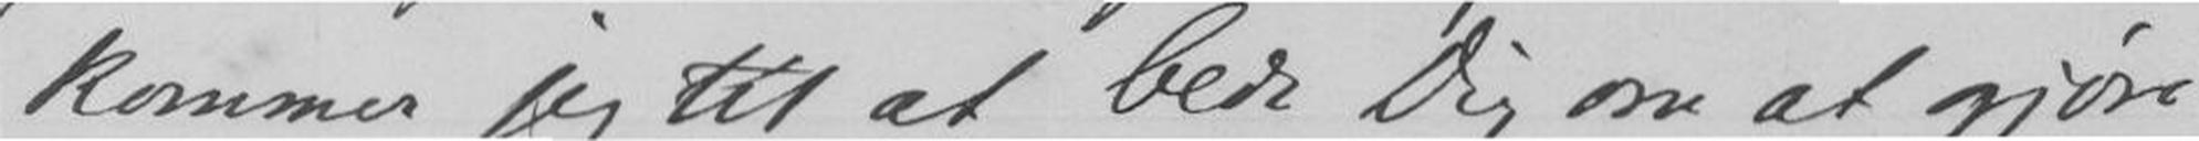kommer jeg til at bede Dig om at gjøre
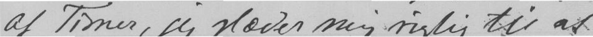af Timer, jeg glæder mig rigtig til at
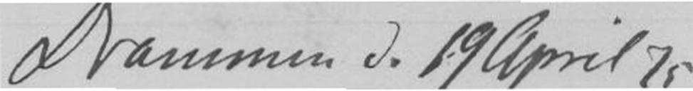Drammen d. 19 April 75
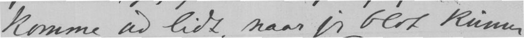komme ud lidt naar jeg blot kunne
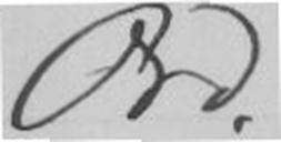Ord.
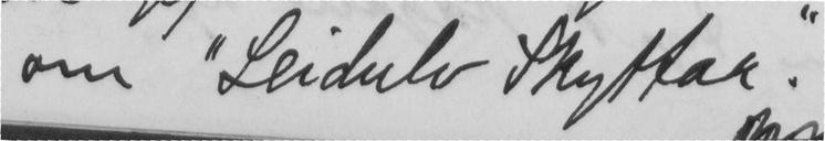om "Leidulv Skyttar."
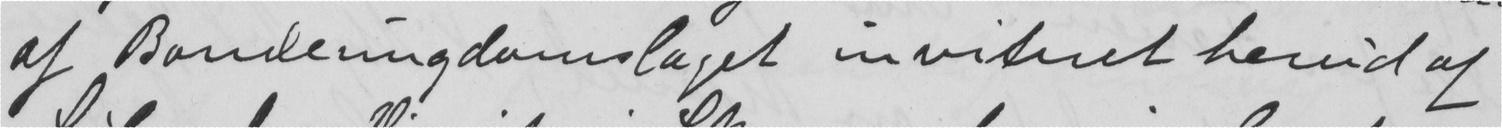af Bondeungdomslaget inviteret herud af
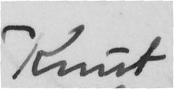Knut
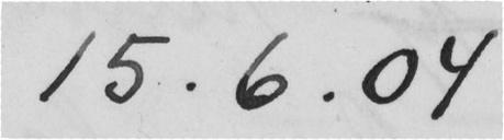15.6.04
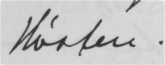Høsten.
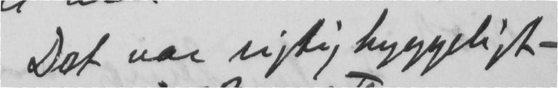Det var rigtig hyggeligt -
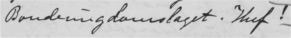Bondeungdomslaget. Huf!
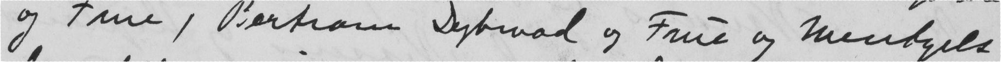og Frue, Bertram Dybwad og Frue og Wentzels
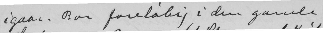igaar. Bor foreløbig i den gamle
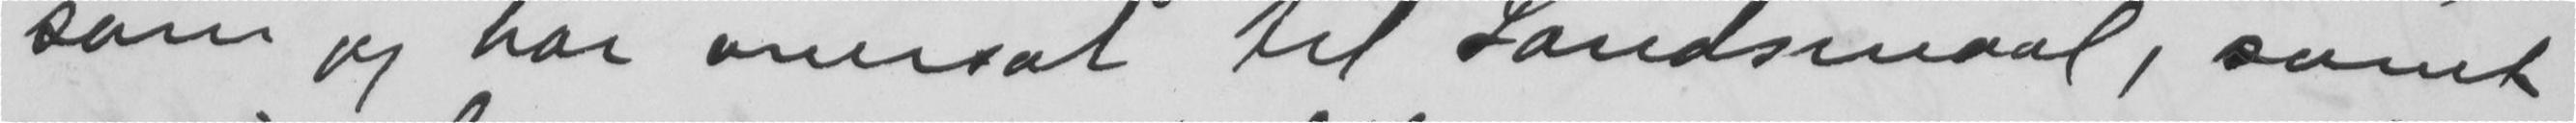som jeg har oversat til Landsmaal, samt
